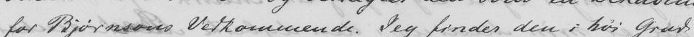for Bjørnsons Vedkommende. Jeg finder den i høi Grad
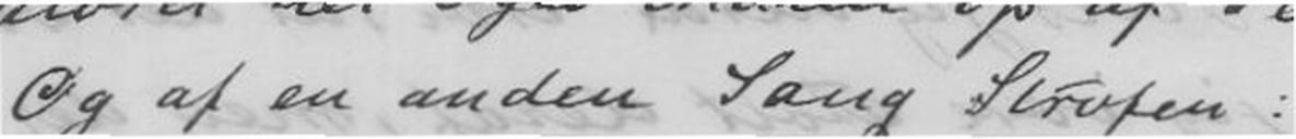Og af en anden Sang Strofen:
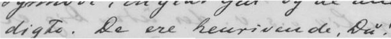digte. De ere henrivende, Du!
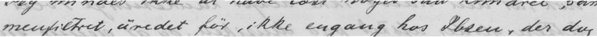menfiltret, uredet før, ikke engang hos Ibsen, der dog
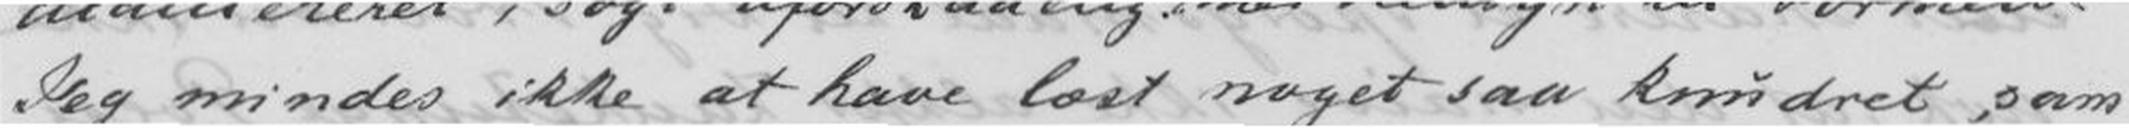Jeg mindes ikke at have læst noget saa knudret sam-
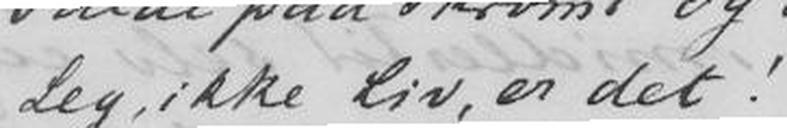Leg ikke Liv, er det!
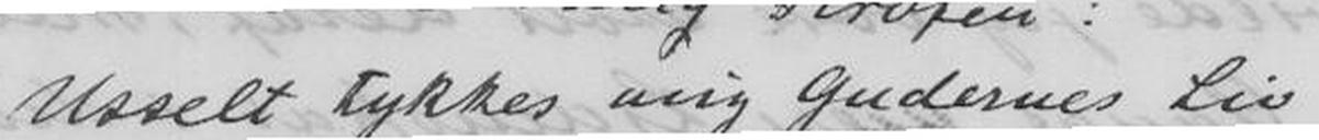Usselt tykkes mig Gudernes Liv
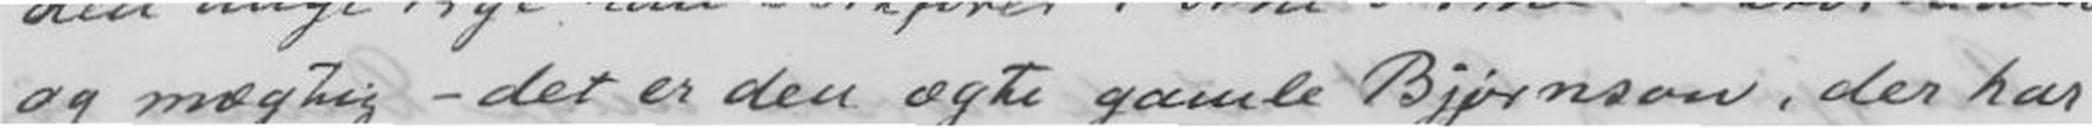og mægtig - det er den ægte gamle Bjørnson, der har
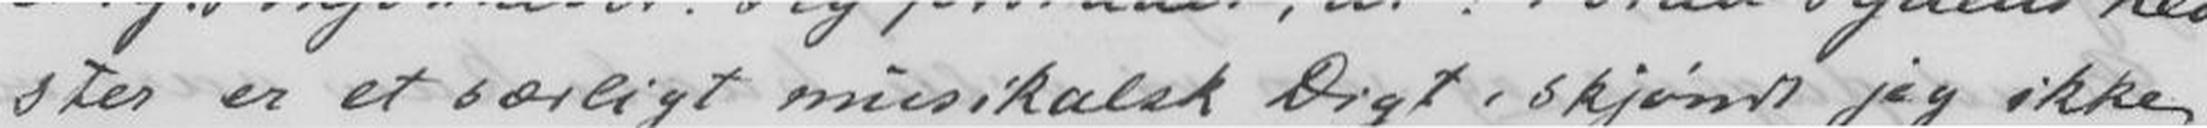ster er et særligt musikalsk Digt, skjønt jeg ikke
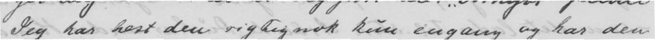Jeg har lest den rigtignok engang og har den
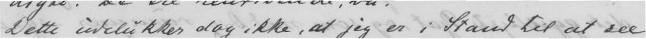Dette udelukker dog ikke, at jeg er i Stand til at see
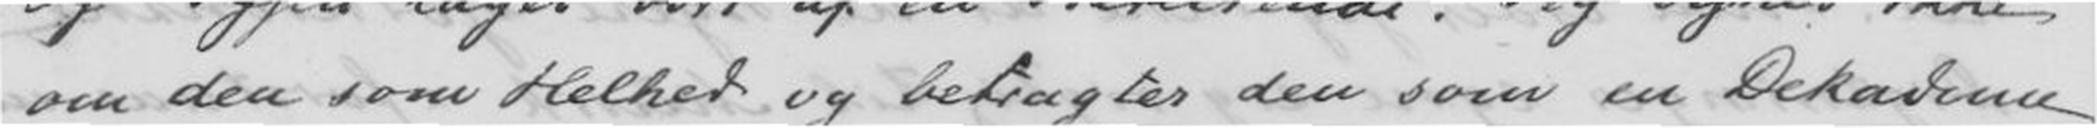om den som Helhed og betragter den som en Dekademe
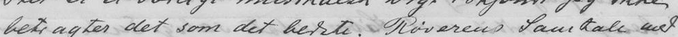betragter det som det bedste. Røverens Samtale med
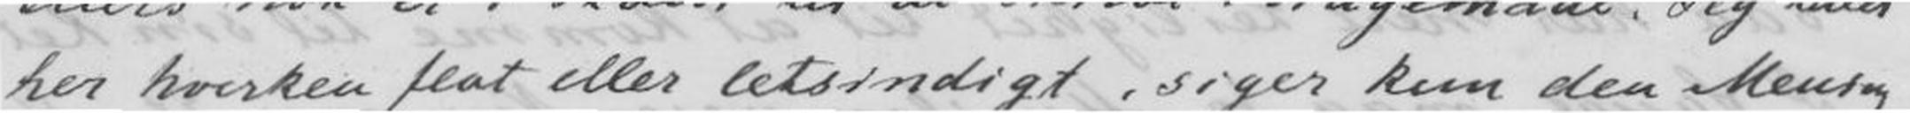her hverken flad eller letsindigt, siger kun den Mening
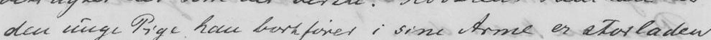den unge Pige han bortføres i sine Arme, er storladen
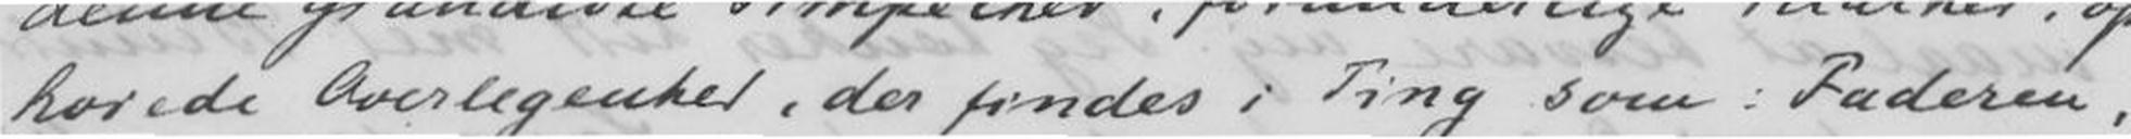høiede Overlegenhed, der findes i Ting som: Faderen,
